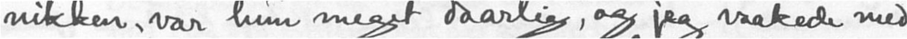nikken, var hun meget daarlig, og jeg vaakede med
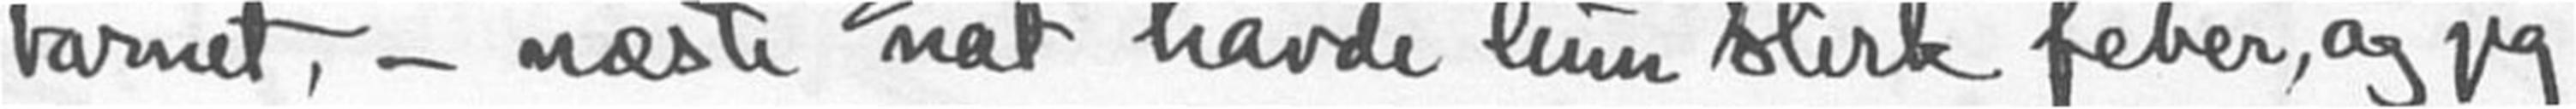barnet, - næste nat havde hun sterk feber, og jeg
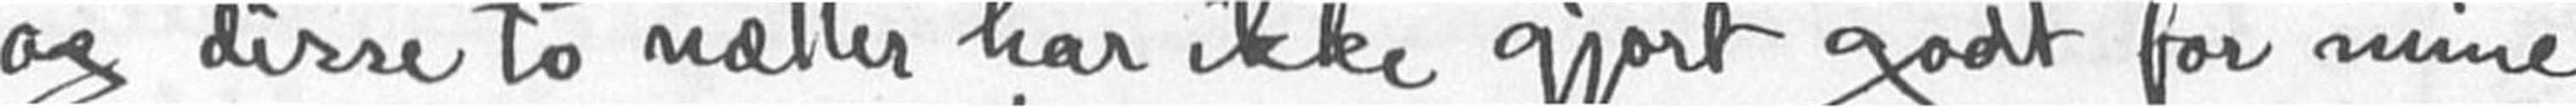og disse to nætter har ikke gjort godt for mine
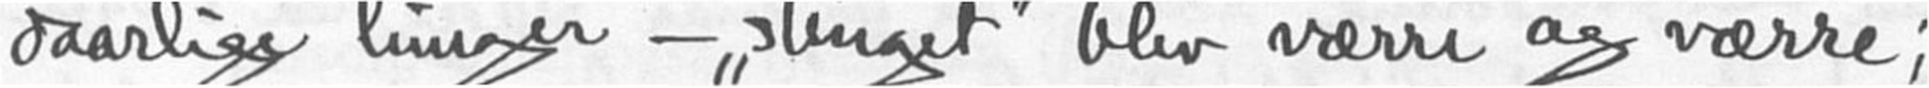daarlige lunger - "stinget" blev værre og værre; -
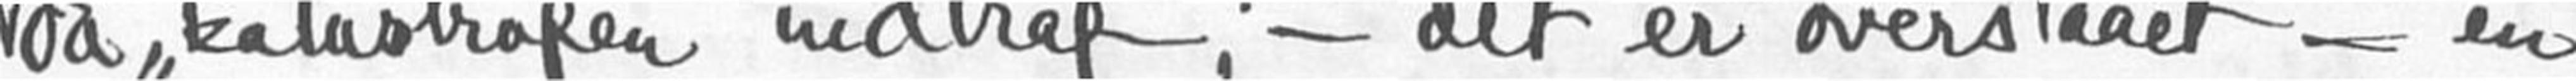da "katastrofen" indtraf; - det er overstaaet - en
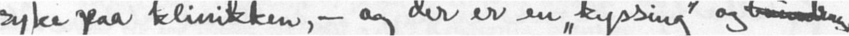syke paa klinikken, - og der er en "kyssing" og
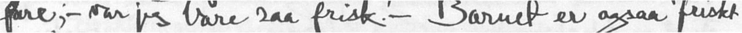fare; - var jeg bare saa frisk! - Barnet er ogsaa friskt
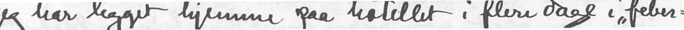jeg har ligget hjemme paa hotellet i flere dage i "feber-
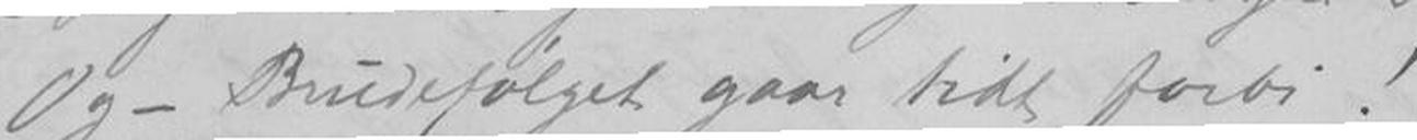Og - Brudefølget gaar tidt forbi!
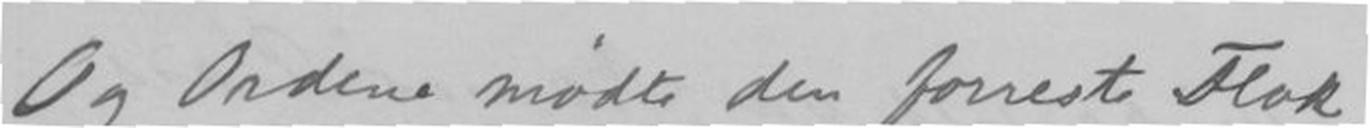Og Ordene mødte den forreste Flok,
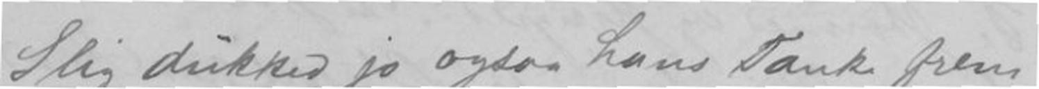Slig dukked jo ogsaa hans Tanke frem,
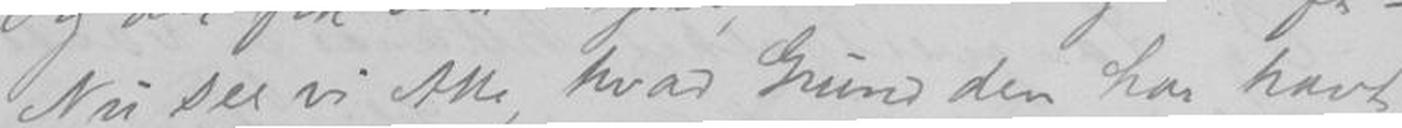Nu ser vi Alle, hvad Grund den har havt,
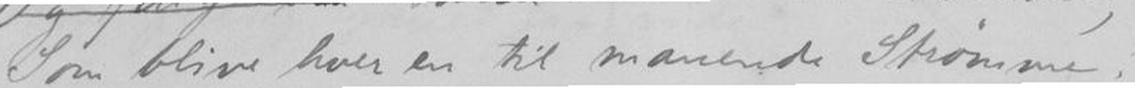Som blive hver en til manende Strømme!
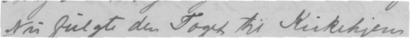Nu fulgte den Toget til Kirkehjem,
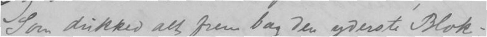Som dukked alt frem bag den yderste Blok;
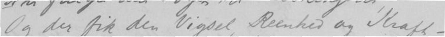Og der fik den Vigsel, Reenhed og Kraft -
